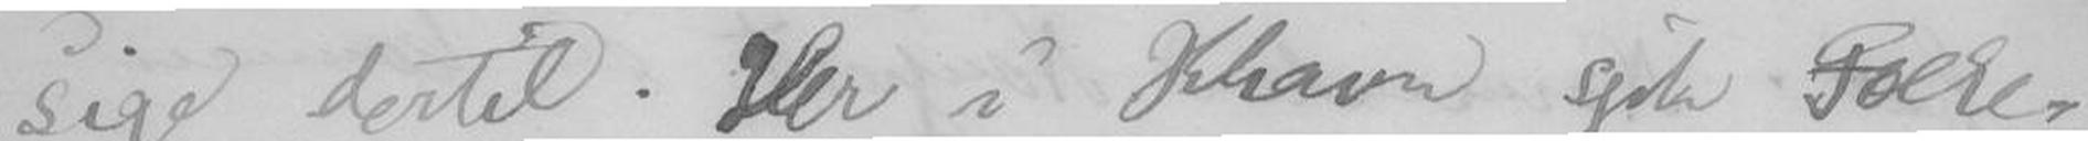sige dertil. Her i Khavn gik Folke-
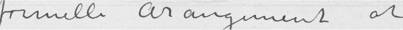formelle Arangement at
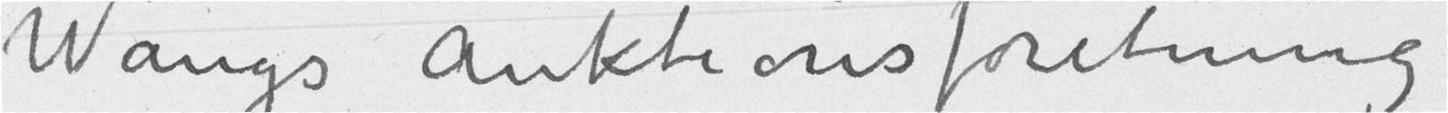Wangs Auktionsforretning
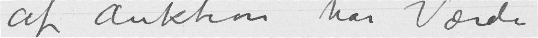af Auktion har Værdi
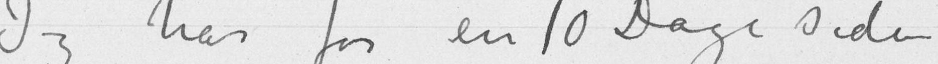Jeg har for en 10 Dage siden
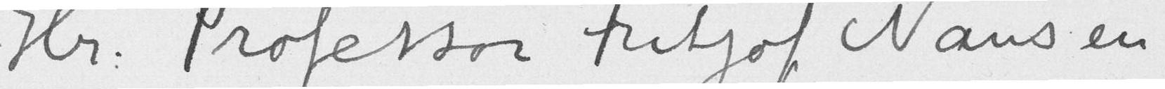Hr. Professor Fritjof Nansen
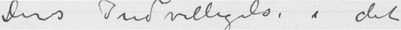Deres Indvilligelse i det
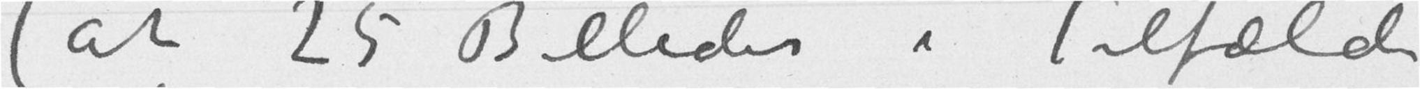(at 25 Billeder i Tilfælde
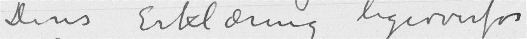Deres Erklæring ligeoverfor
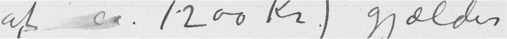af ca. 1200 Kr.) gjælder
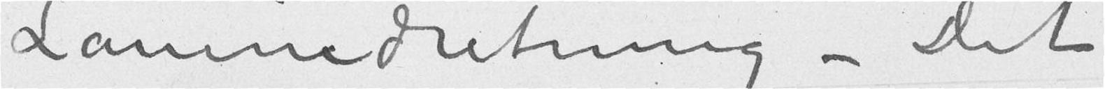Låneindretning – Det bliver
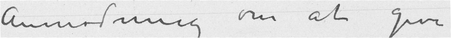Anmodning om at give
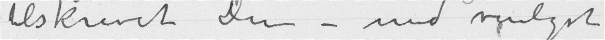tilskrevet Dem – med venligst
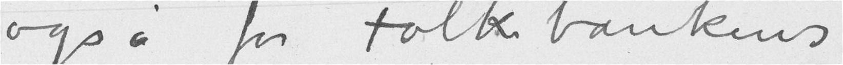også for Folkebankens
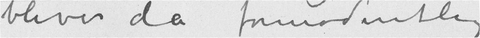da formodentlig
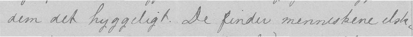dem det hyggeligt. De finder menneskene elsk-
